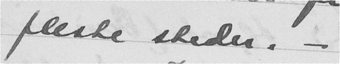fleste steder. -
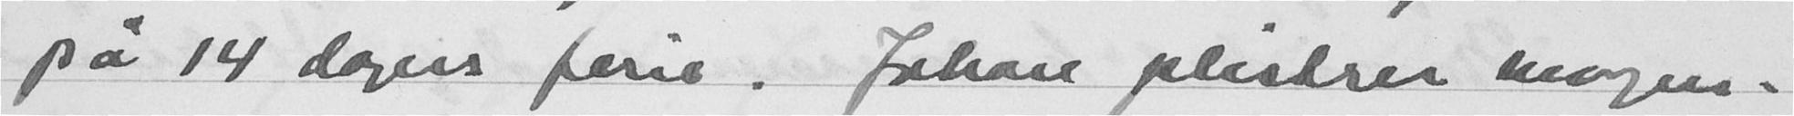på 14 dages ferie. Johan plistrer morgen-
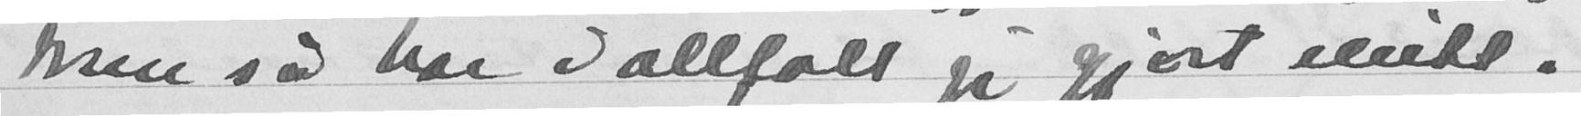Men så har iallfall jeg gjort mitt.
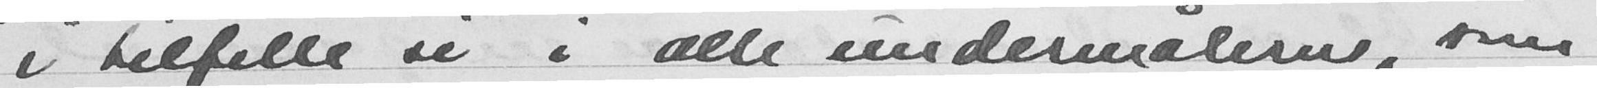i tilfelle si i alle undermålerne, som
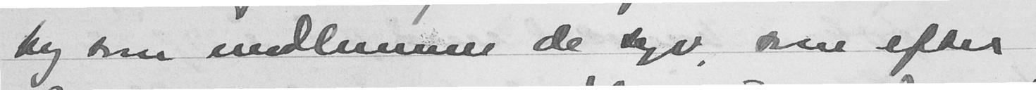by som medlemme de syv, som efter
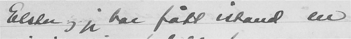Elster og jeg har fått istand en
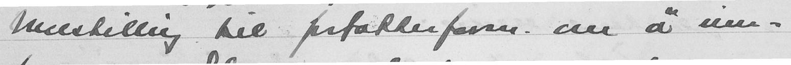innstilling til forfatterforen. om å inn-
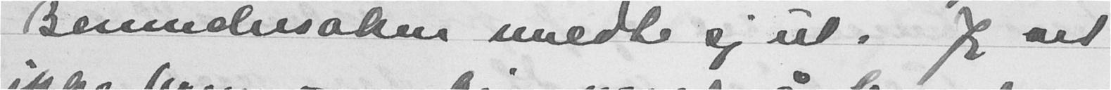Bennechesaken meldte sig ut. Jeg vet
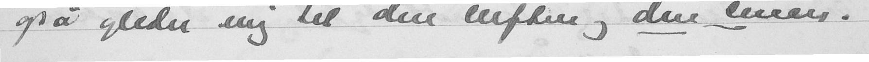også gleder mig til den luften og den søvnen.
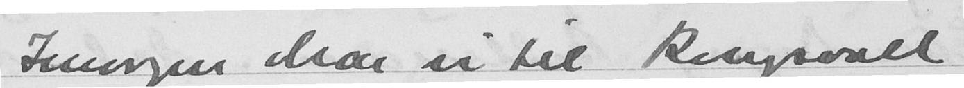Imorgen drar vi til Kongsvoll
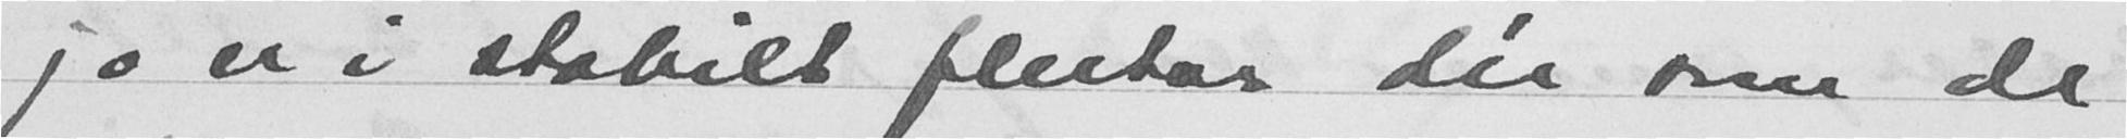jo er i stabilt flertall dér som de
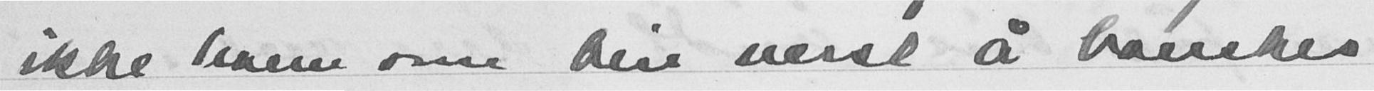ikke hvem om blir verst å hanskes
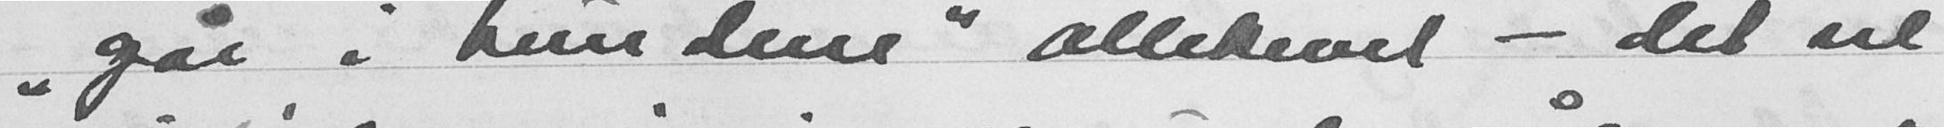"går i hundene" allikevel - det vil
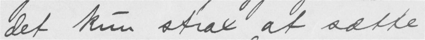Det kun strax at sætte
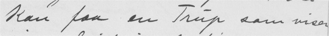kan faa en Trup som viser
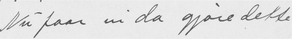Nu faar vi da gjøre dette
